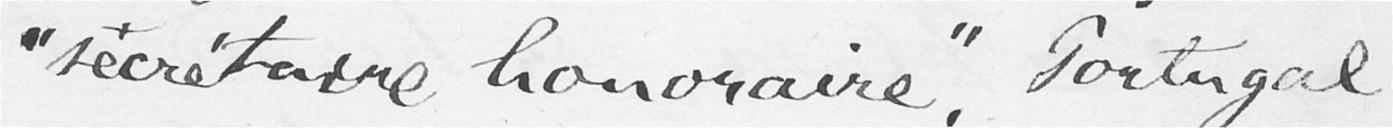"secrétaire honoraire", Portugal
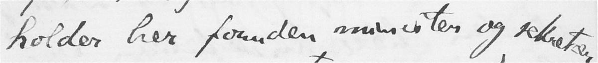holder her foruden minister og sekretær,
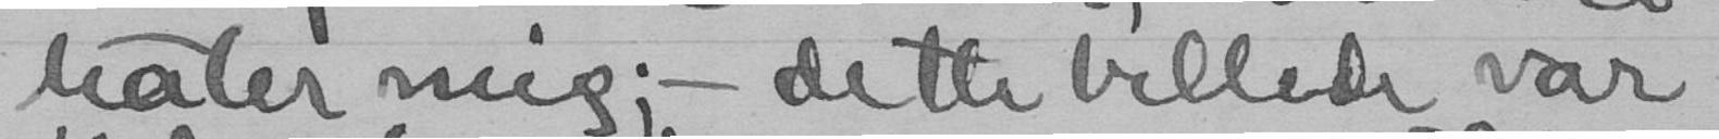hater mig; - dette billede var
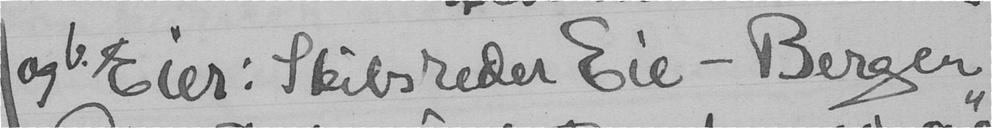og b. Eier: Skibsreder Eie - Bergen
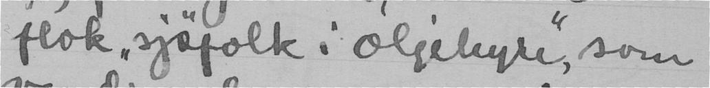flok "sjøfolk i oljehyre", som
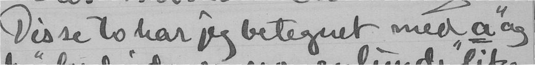Disse to har jeg betegnet med "a" og
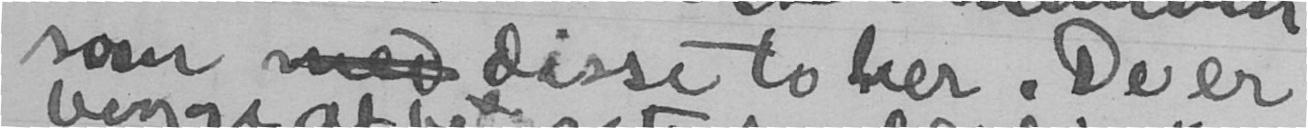som med disse to her. De er
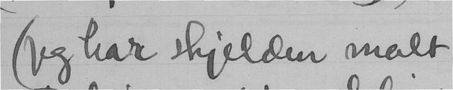(jeg har skjelden malt
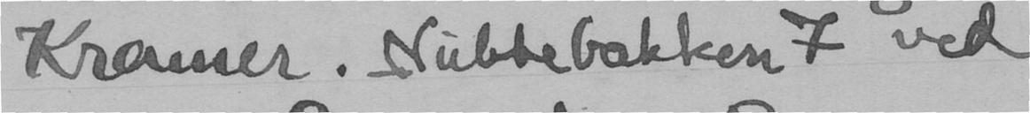Kramer. Nubbebakken 7 ved
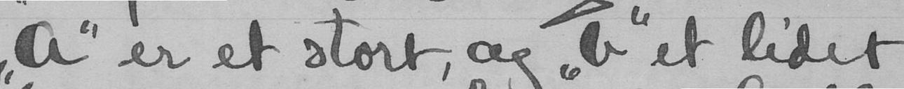"a" er et stort, og "b" et lidet
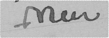Men
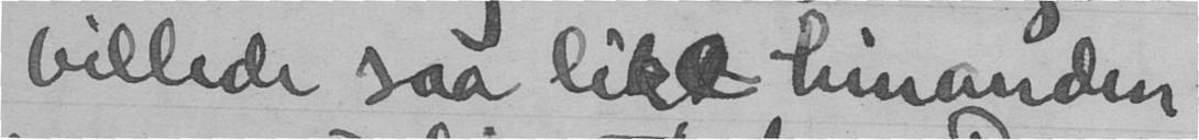billede saa likt hinanden
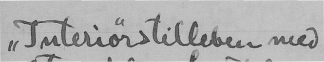"Interiorstilleben med
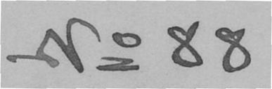No 88
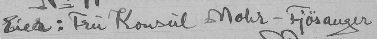Eier: Fru Konsul Mohr - Fjøsauger
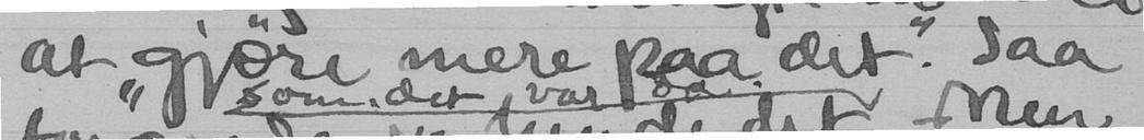at "gjøre mere paa det". Saa
